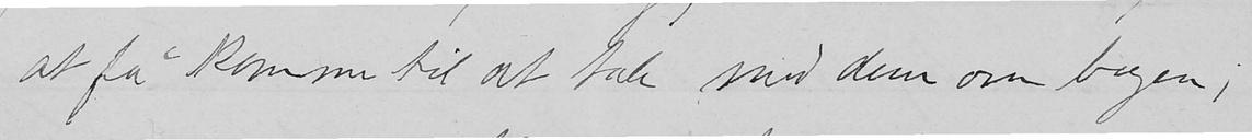at få komme til at tale medd dem om bogen,
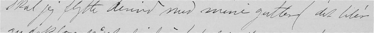skal jeg flytte derind med mine gutter (det blir
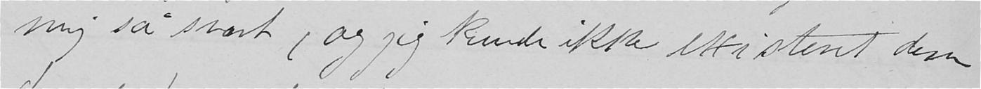mig så svært, og jeg kunde ikke existeret dem
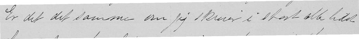Er det det samme om jeg skriver i stort eller lidet
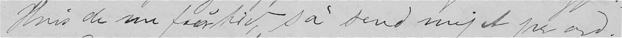Hvis de nu får tid, så send mig et par ord.
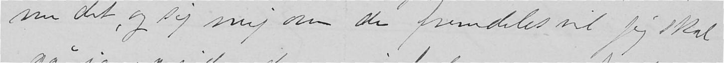om det, og sig mig om de fremdelets vil jeg skal
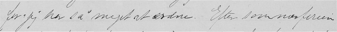for jeg har så meget at ordne. Efter sommerferien
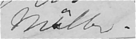Müller.
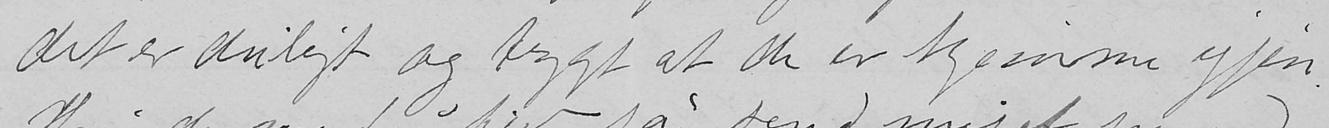det er deiligt og trygt at de er hjemme igjen.
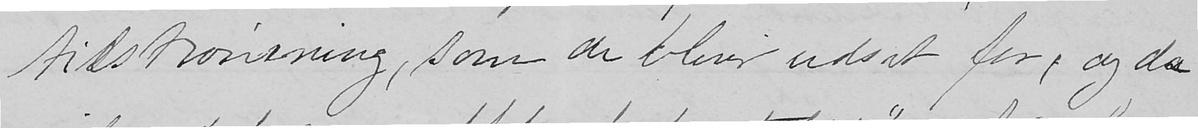tilstrømning, som de bliver udsat for, og da
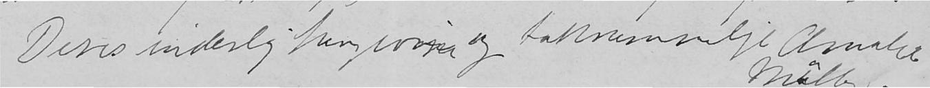Deres inderlig hengivne og taknemmelige Amalie
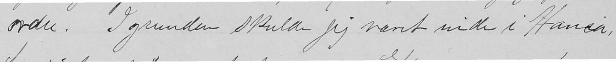ordre. Igrunden skulde jeg været inde i Xania,
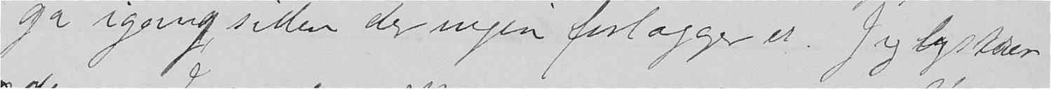gå igang, siden der ingen forlægger er. Jeg lystrer
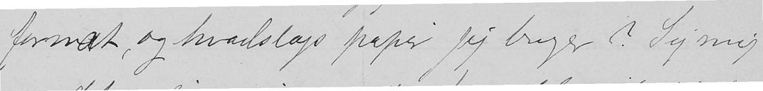format, og hvadslags papir jeg bruger? Sig mig
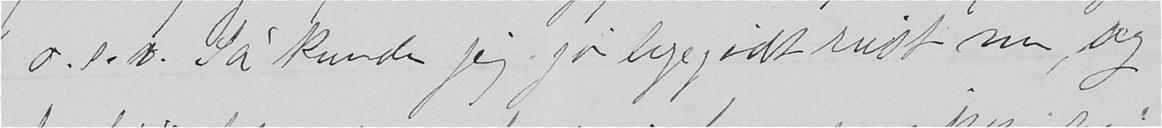o.s.v. Så kunde jeg jo ligegodt reist nu, og
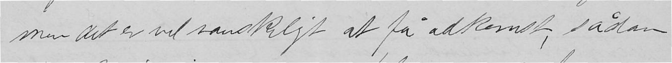men det er vel vanskeligt at få adkomst, sådan
